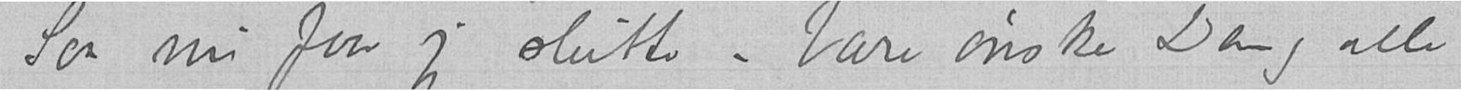Saa nu faar jeg slutte – bare ønske Dem og alle
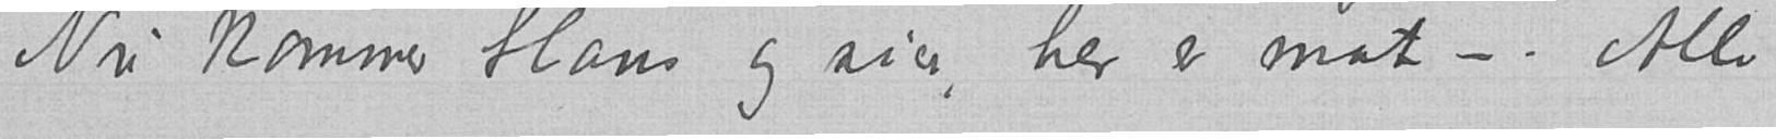Nu kommer Hans og sier her er mat –. Alle
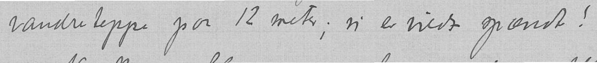vandreteppe paa 12 meter; vi er vildt spændt!
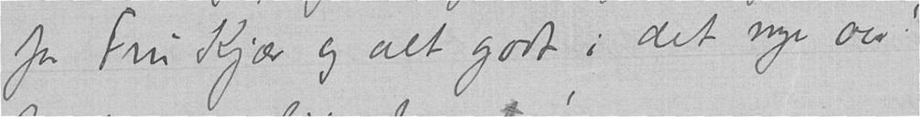for Fru Kjær og alt godt i det nye aar!
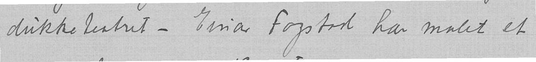dukketeatret - Einar Fogstad har malet et
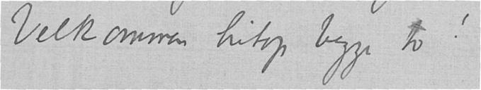Velkommen hitop begge to!
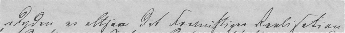Dyden er altsaa det Fornuftiges Realisation paa
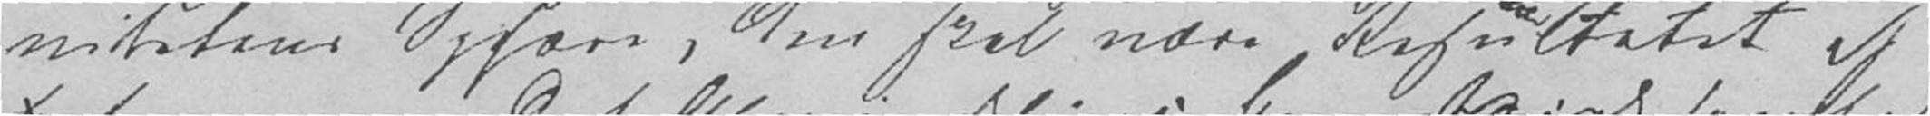vitetens Sphære, den skal være Resultatet af
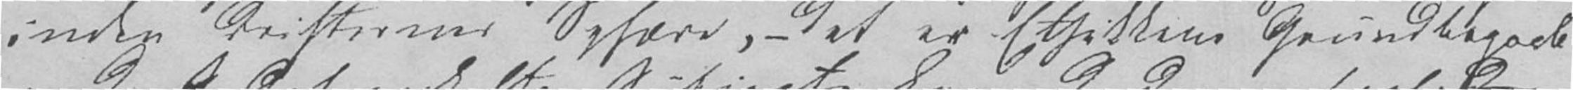inden Drifternes Sphære, det er Ethikkens Grundbegreb.
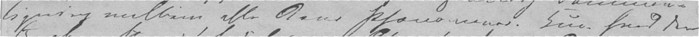ligning mellem alle dens Phænomener. Kun hvad den
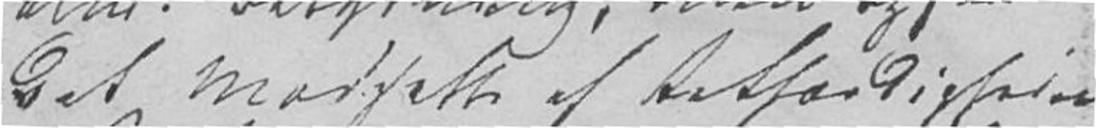Det Modsatte af Retfærdigheden
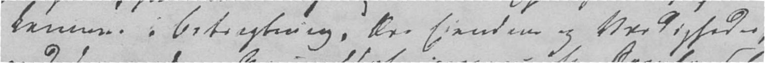kommer i Betragtning. Ære, Eiendom og Værdigheder
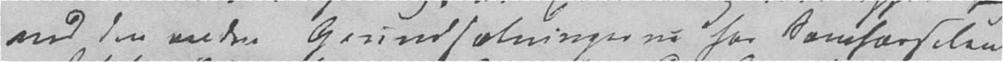ved den andre Grundsætningerne for Samfærdselen
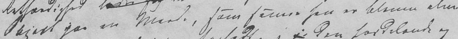Object paa en Maade, som senere hen er bleven alm.
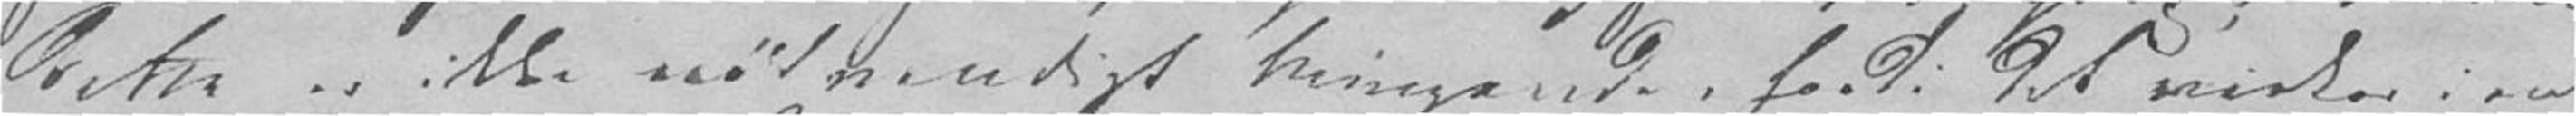dette er ikke nødvendigt tvingende, fordi det virker i en
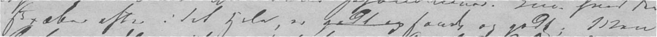stræber efter i det Hele, er godt og sandt og godt. Men
